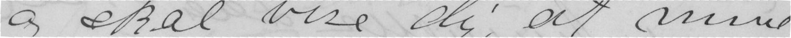og skal vise dig, at mine
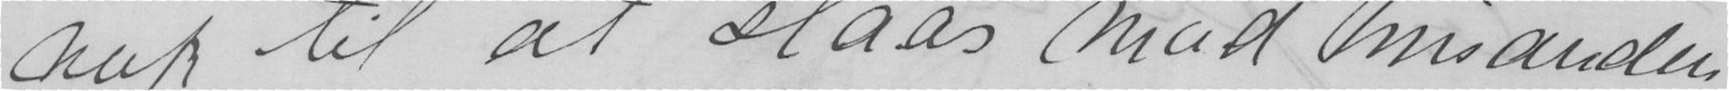nok til at slaas mod hinanden
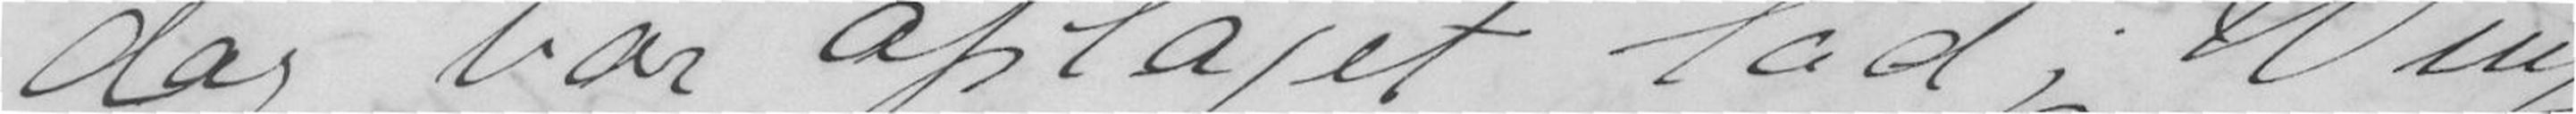dag var optaget, lod jeg Winje
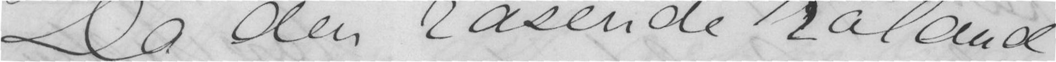Da den rasende Roland
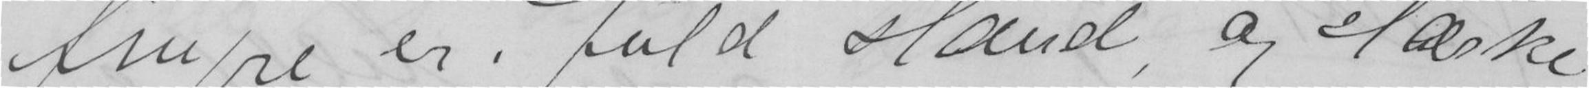fingre er, fuld stand, og stærke
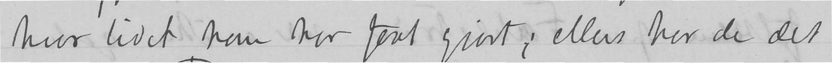hvor lidet han har faat gjort; ellers har de det
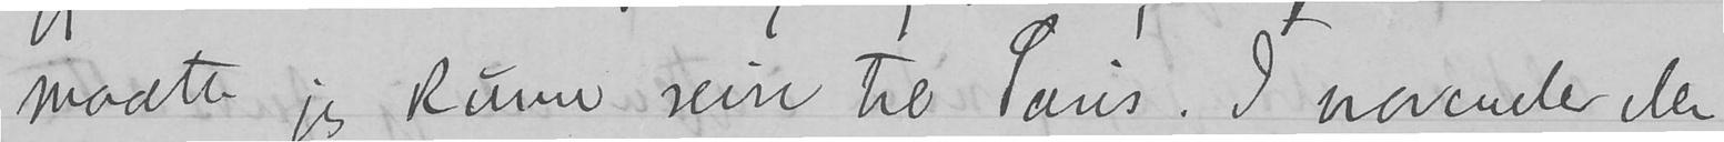maatte jeg kunne reise til Paris. I november eller
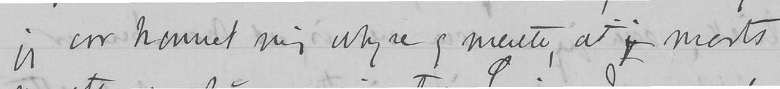jeg var kommet mig uhyre og mente, at jeg marts
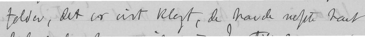folder, det er vist klogt, de havde neppe havt
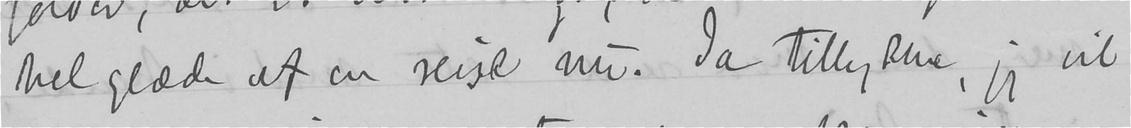hel glæde af en reise nu. Ja tillykke, jeg vil
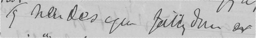og hendes egen fattigdom er
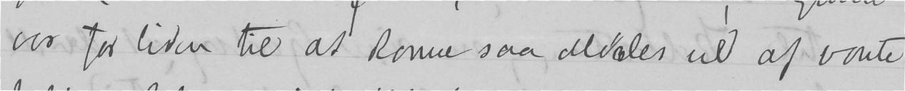var for liden til at komme saa aldeles ud af vante
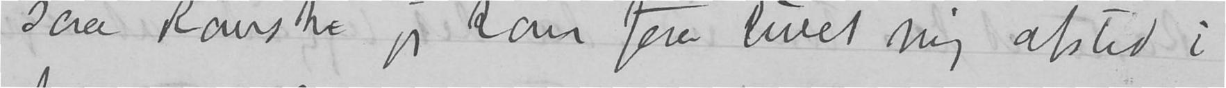saa kanske jeg kan faa livet mig afsted i
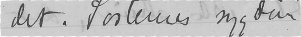det. Søstrenes sygdom
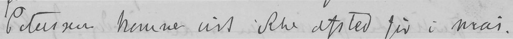Peterssen kommer vist ikke afsted før i mai.
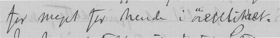for meget for hende i øieblikket.
